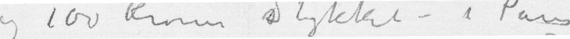og 100 Kroner Stykket – i Paris
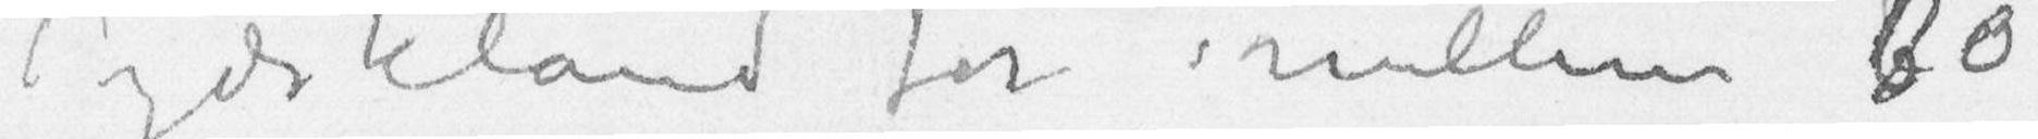Tydskland for mellem 60
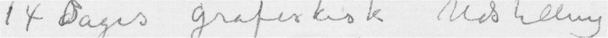14 Dages grafisk Udstilling
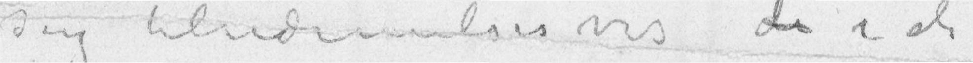sig tilnærmelsesvis de i de
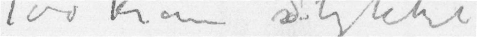100 Kroner Stykket
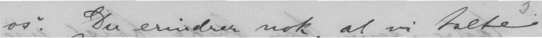os. Du erindrer nok, at vi talte
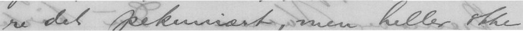re det pekuniært, men heller ikke
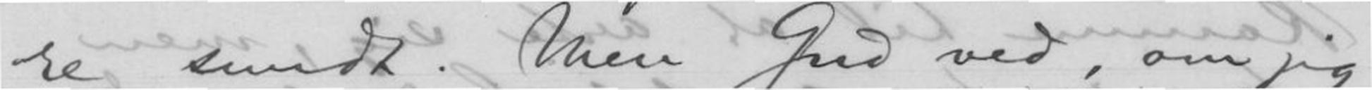re sundt. Men Gud ved, om jeg
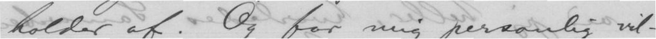holder af. Og for mig personlig vil-
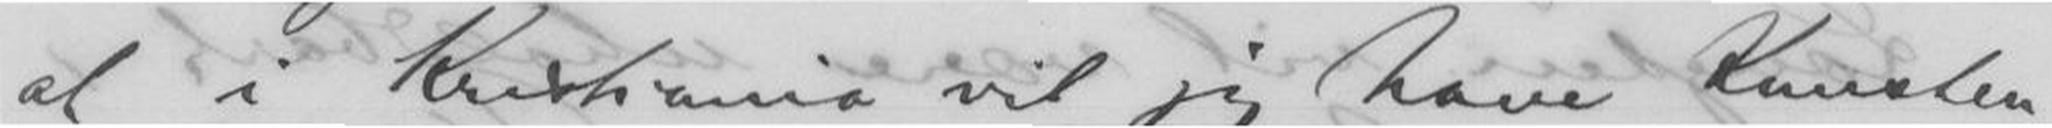at i Kristiania vil jeg have Kunsten
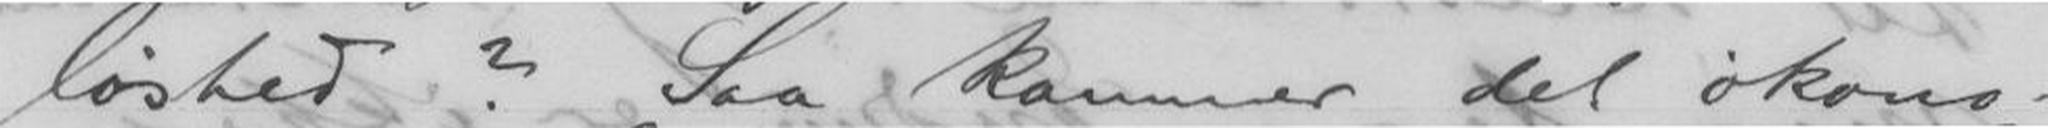løshed? Saa kommer det økono-
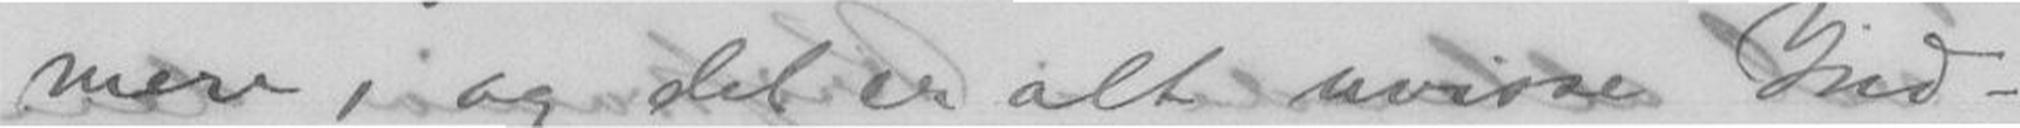mere, og det er alt uvisse Ind-
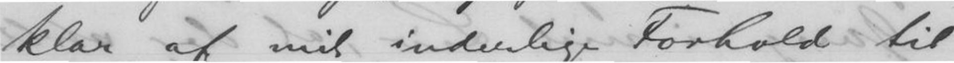klar af mit inderlige Forhold til
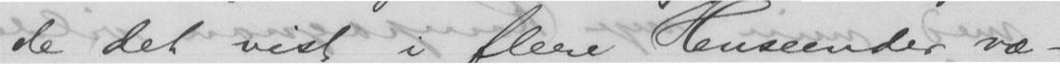de det vist i flere Henseender væ-
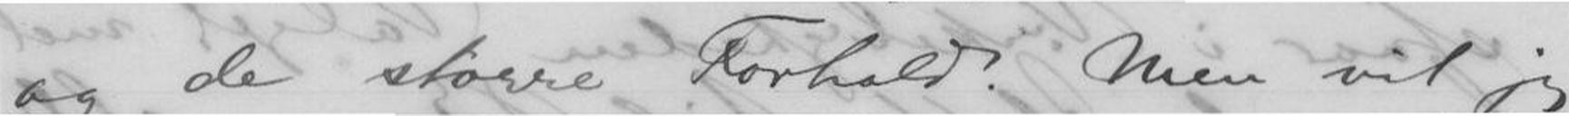og de større Forhold. Men vil jeg
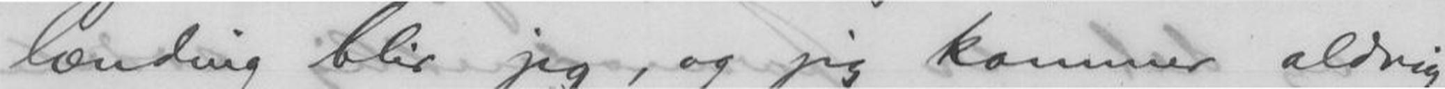lænding blir jeg, og jeg kommer aldrig
